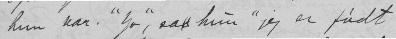hun var. "Jo", sa hun "jeg er født
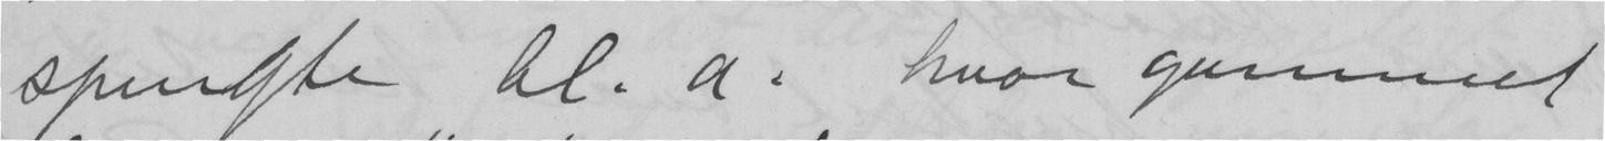spurgte bl. a. hvor gammel
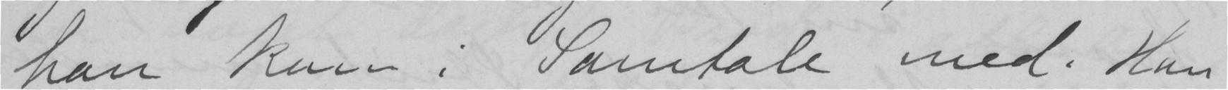han kom i Samtale med. Han
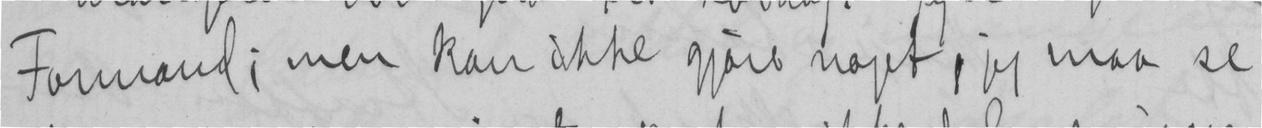Formand; men kan ikke gjøre noget, jeg maa se
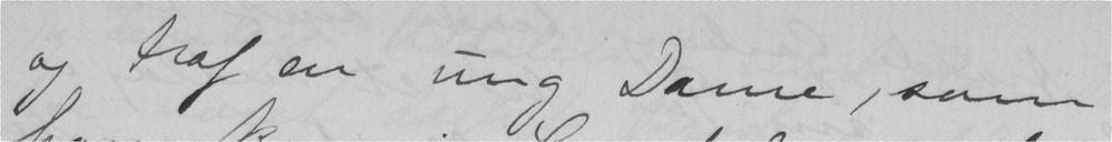og traf en ung Dame, som
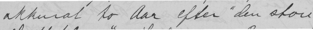akkurat to Aar efter "den store
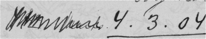4.3.04
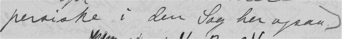persiske i den Sag her ogsaa.
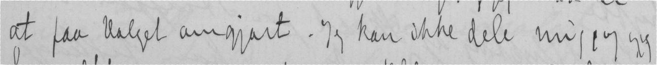at faa Valget omgjort. Jeg kan ikke dele mig, og jeg
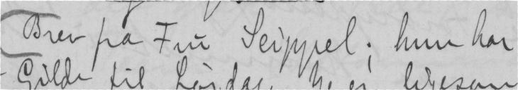Brev fra Fru Seippel; hun har
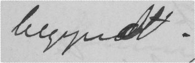begyndt.
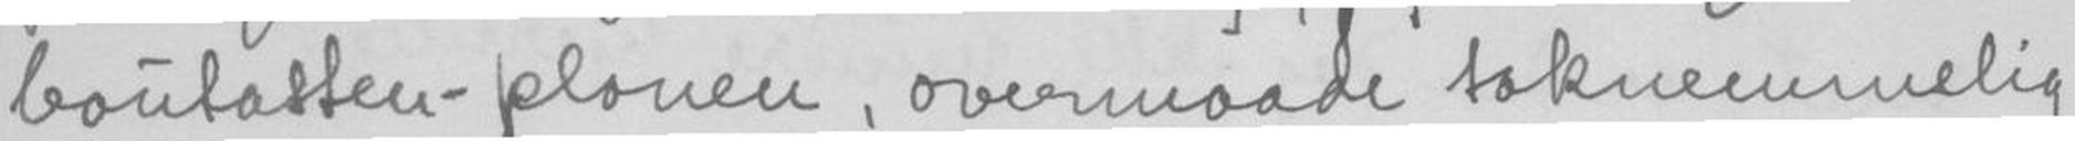bautasten-planen, overmaade taknemmelig
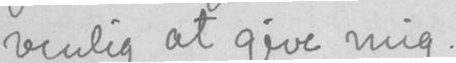venlig at give mig.
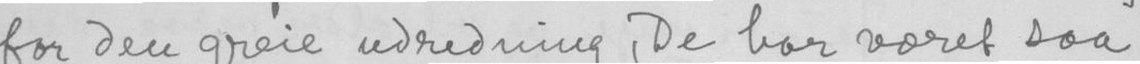for den greie udredning, de har været saa
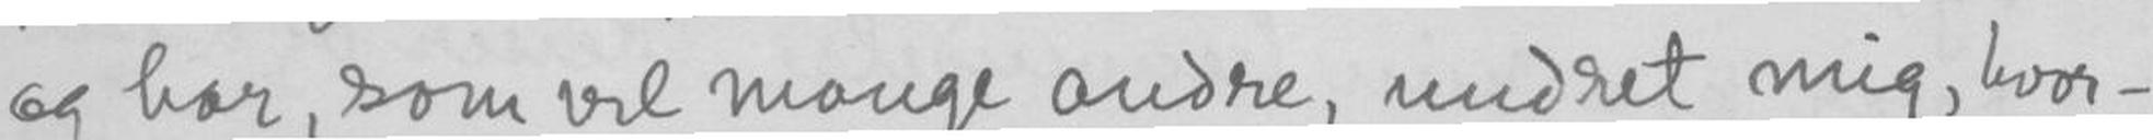og har, som vel mange andre, undret mig, hvor-
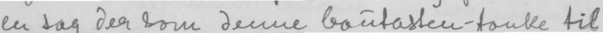en sag, der som denne bautasten-tanke til-
